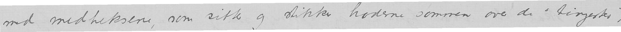med medhekserne, som sitter og stikker hoderne sammen over de «tingester
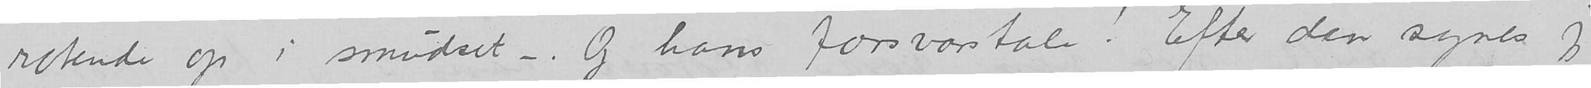rotende op i smudset –. Og hans forsvarstale! Efter den synes jeg
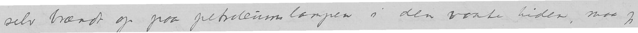selv brændt op paa petroleumslampen i den vaate tiden, naar jeg
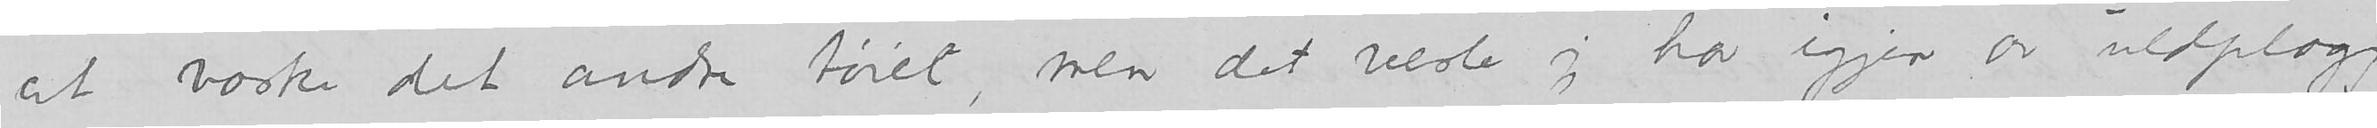at vaske det andre tøiet, men det vesle jeg har igjen av uldplagg,
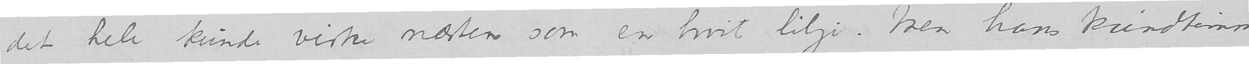det hele kunde virke næsten som en hvit lilje. Men hans fruendtimmer/kundstimmer/kvindtimmer
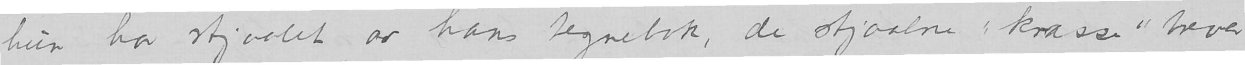», hun har stjaalet av hans tegnebok, de stjaalne «krasse» brever
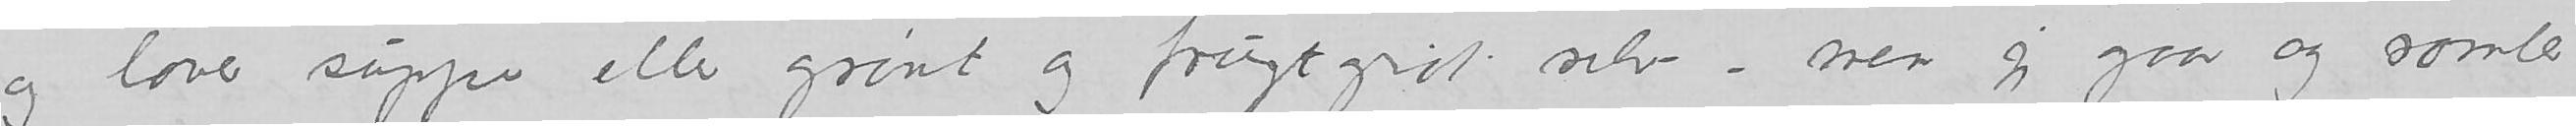og laver supper eller grønt og frugtgrøt selv – men jeg gaar og somler
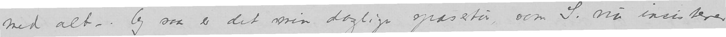med alt –. Og saa er det min daglige spasertur, som S. nu insisterer
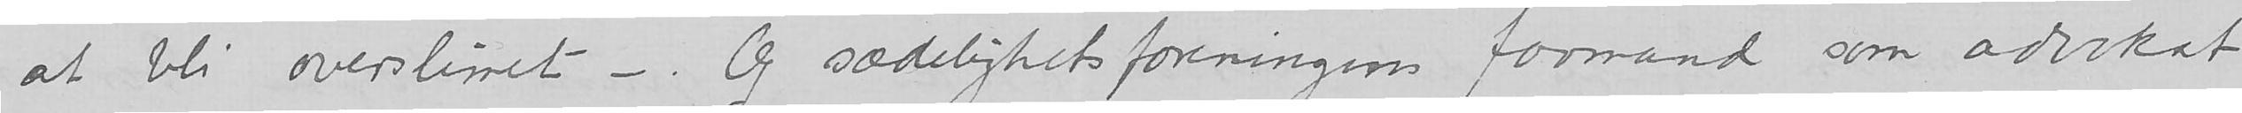at bli overslirret –. Og sædelighetsforeningens formand som advokat
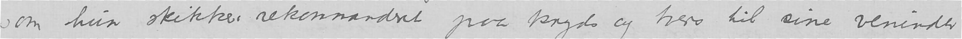som hun skikker rekommanderet paa kryds og tvers til sine veninder,
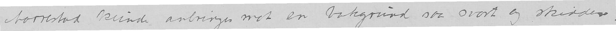Aarrestad kunde anbringes mot en bakgrund saa svart og skidden,
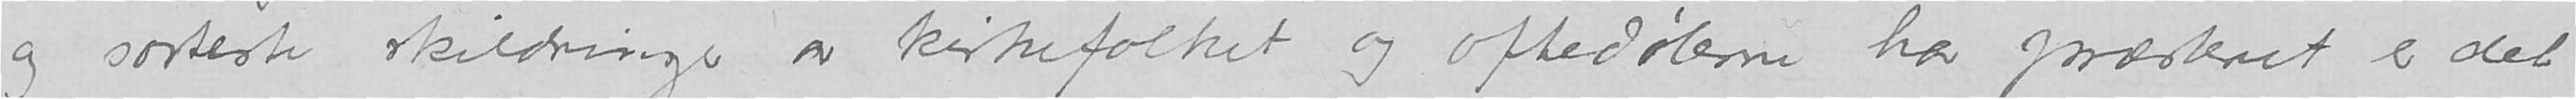og sorteste skildringer av kirkefolket og ofterdølerne har præsteret er det
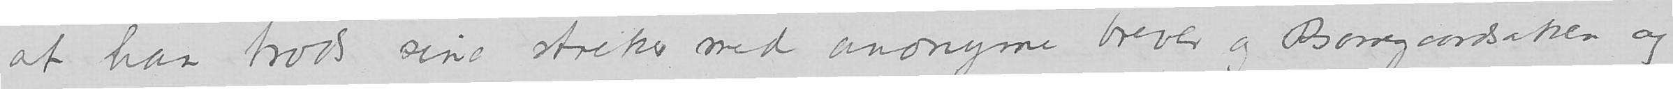at han trods sine streker med anonyme brever og Borregaardsaken og
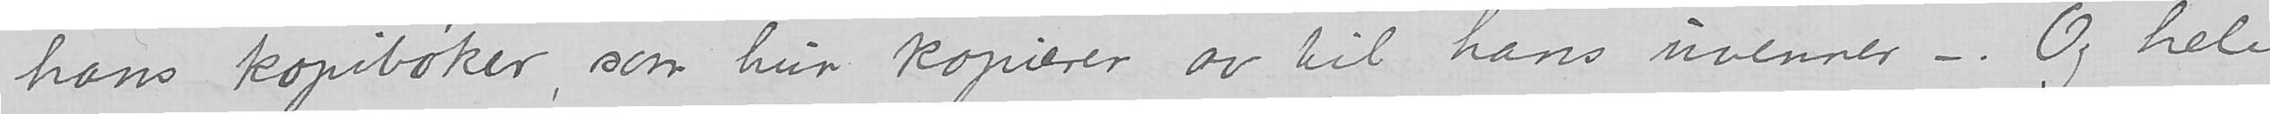hans kopibøker, som hun kopierer av til hans uvenner –. Og hele
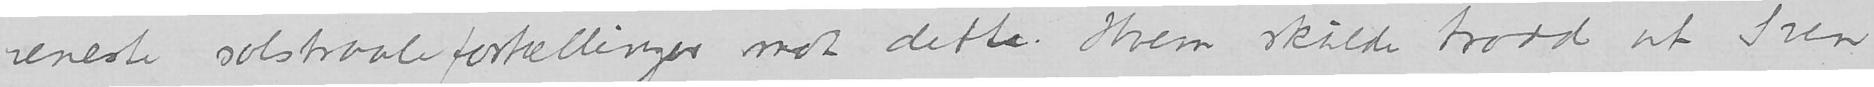reneste solstraalefortellinger mot dette. Hvem skulde trodd at Sven
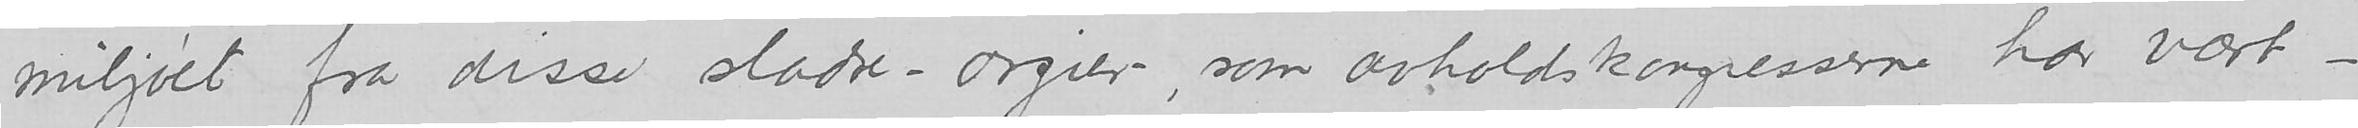miljøet fra disse sladre-orgier, som avholdskongresserne har vært –
